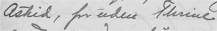Astrid, for uden Thrine
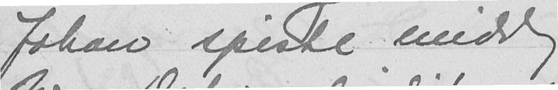Johan spiste middag
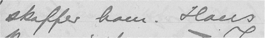skaffer ham. Hans
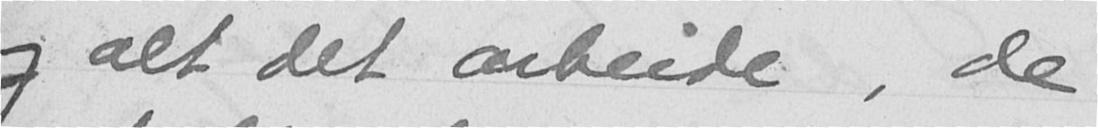og alt det arbeide, de
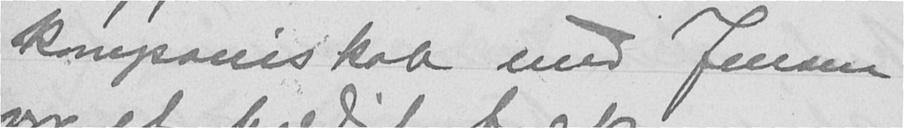kompaniskab med Jensen
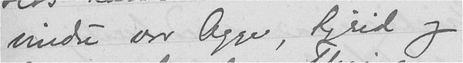vindu var Agga, Sigrid og
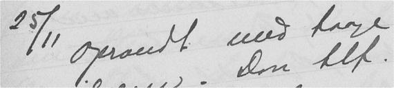25/11 oprandt med taage
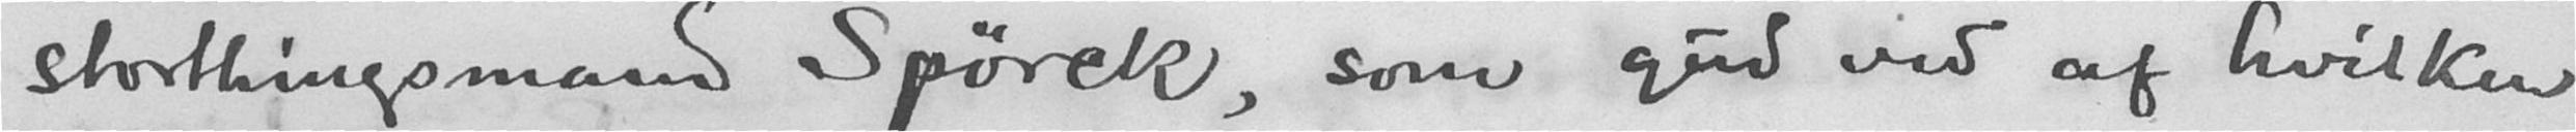storthingsmand Spørck, som gud ved af hvilken
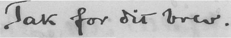Tak for dit brev.
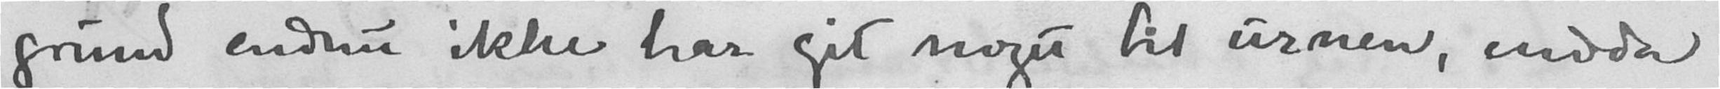grund endnu ikke har git noget til urnen, endda
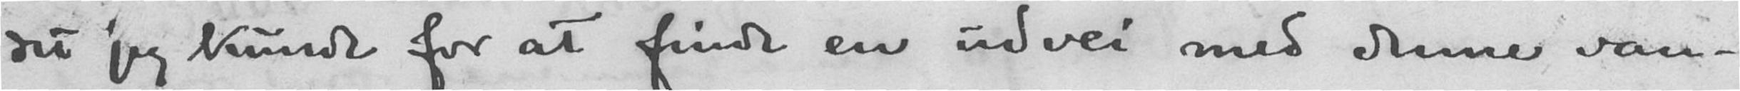det jeg kunde for at finde en udvei med denne van-
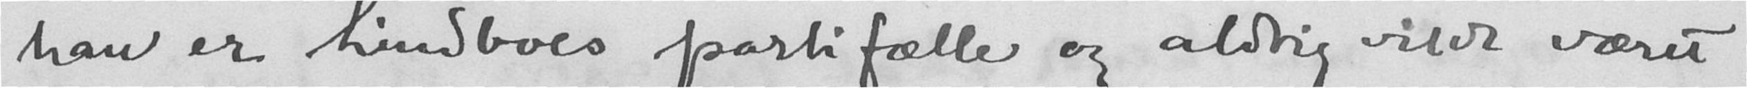han er Lindboes partifælle og aldrig vilde været
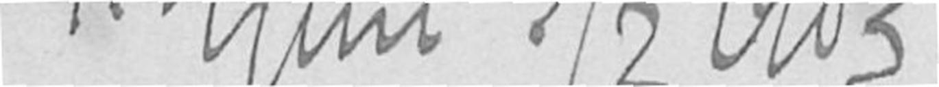T.hjem 3/2 1903
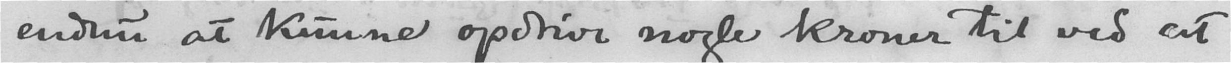endnu at kunne opdrive nogle kroner til ved at
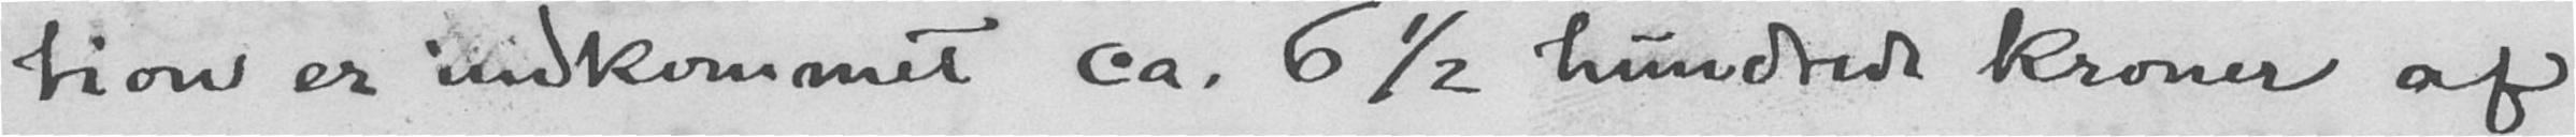tion er indkommet ca. 6½ hundrede kroner af
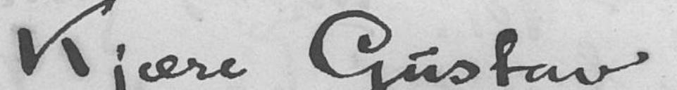Kjære Gustav
